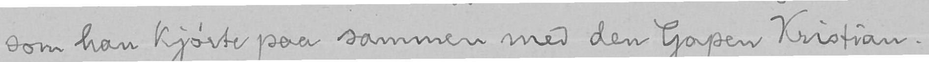som han kjørte paa sammen med den Gapen Kristian.
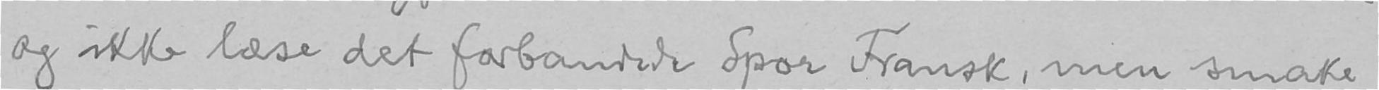og ikke læse det forbandede Spor Fransk, men smake
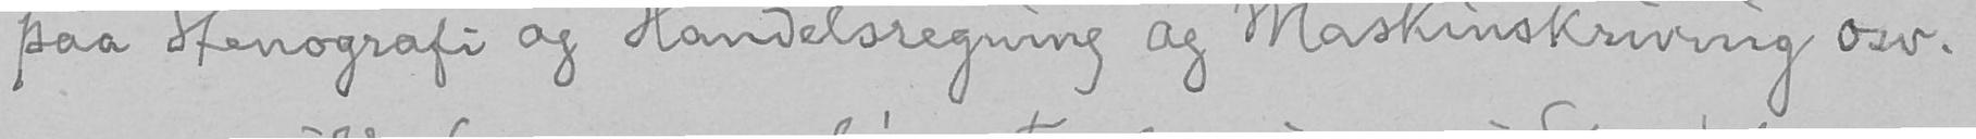paa Stenografi og Handelsregning og Maskinskriving osv.
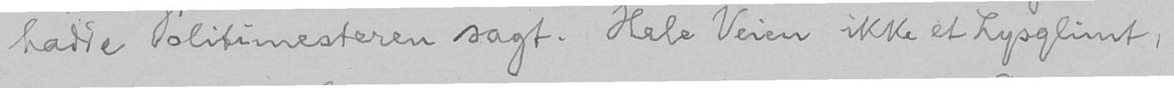hadde Politimesteren sagt. Hele Veien ikke et Lysglimt,
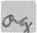og
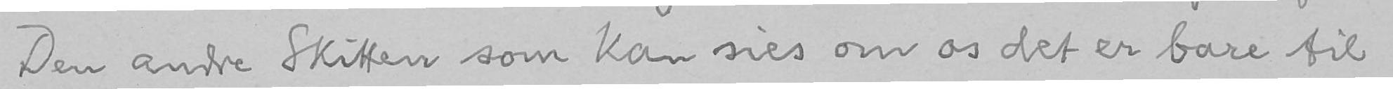Den andre Skitten som kan sies om os det er bare til
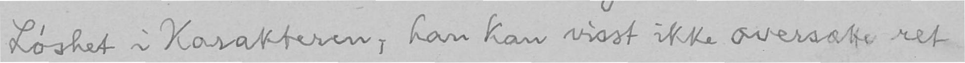Løshet i Karakteren, han kan visst ikke oversætte ret
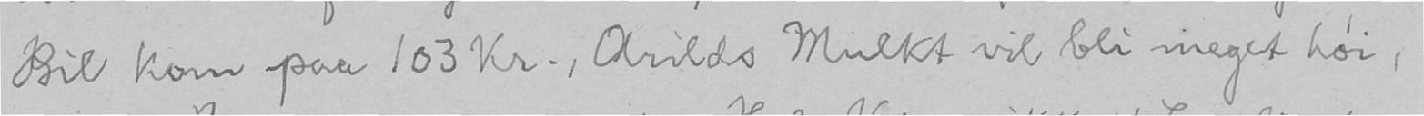Bil kom paa 103 Kr., Arilds Mulkt vil bli meget høi,
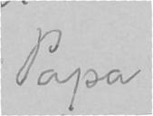Papa
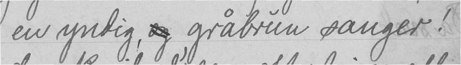en yndig, og gråbrun sanger!
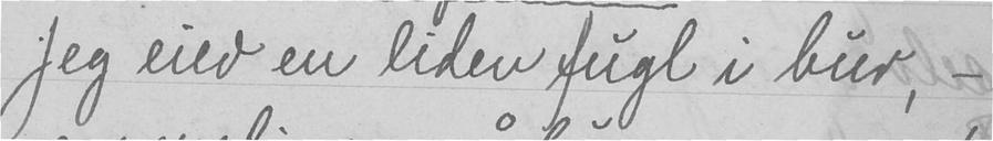Jeg eied en liden fugl i bur, -
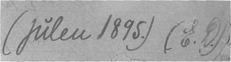(julen 1895.) (E.D.)
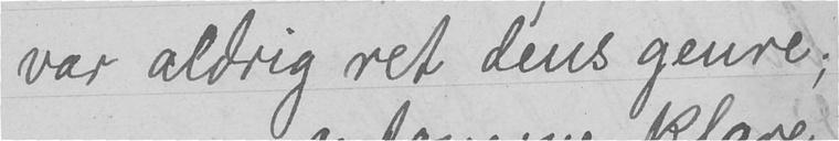var aldrig ret dens genre;
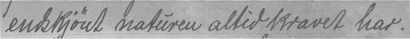endskjønt naturen altid kravet har.
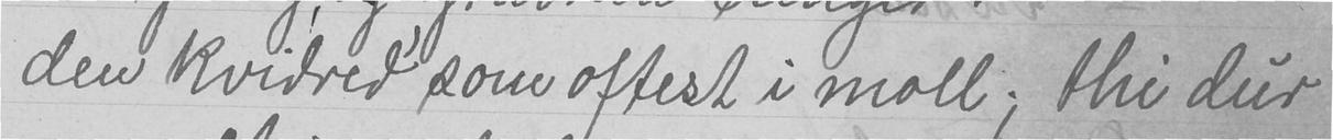den kvidred som oftest i moll, thi dur
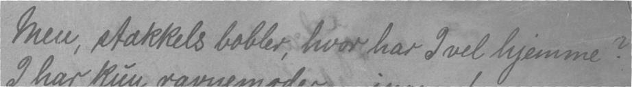Men, stakkels bobler, hvor har I vel hjemme?
